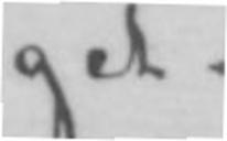get.
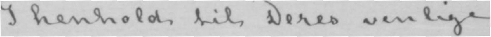I henhold til Deres venlige
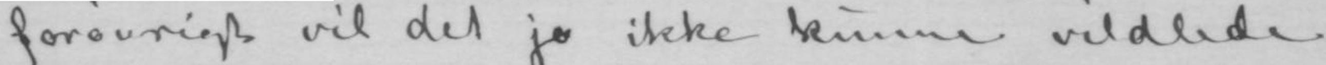forøvrigt vil det jo ikke kunne vildlede
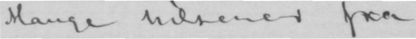Mange hilsener fra
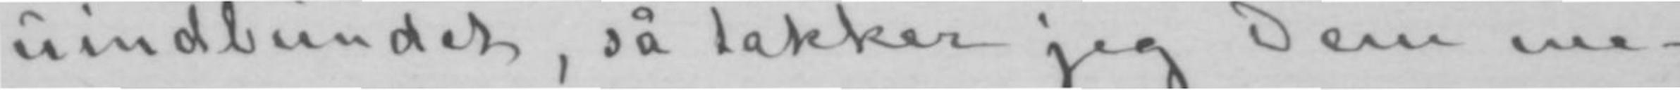uindbundet, så takker jeg Dem me-
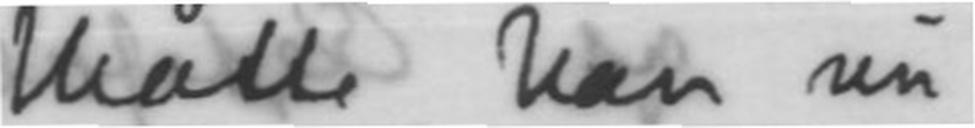Måtte han nu
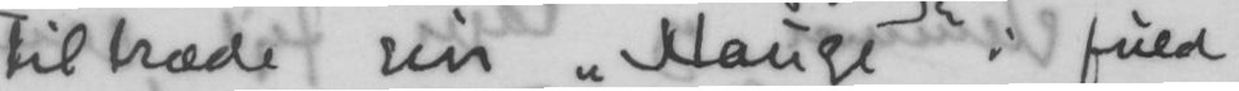tiltræde sin "Hauge" i fuld
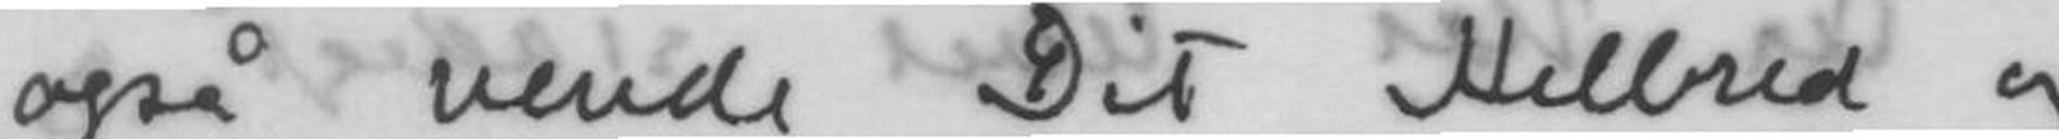også vende Dit Helbred og
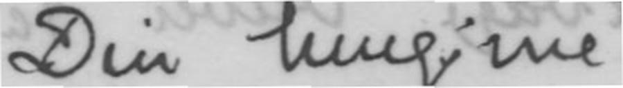Din hengivne
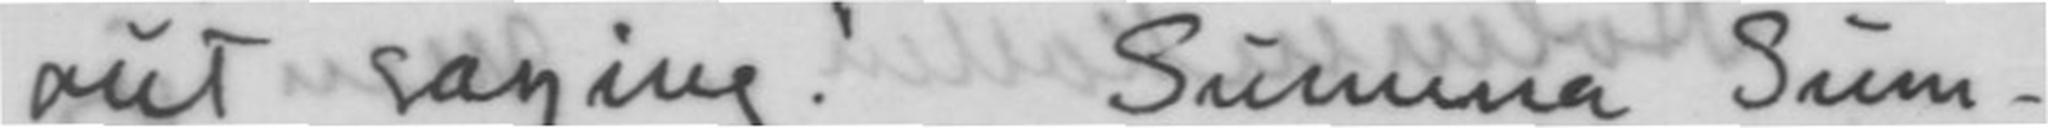out saying. Summa Sum-
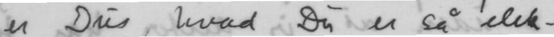er Dus, hvad Du er så elsk-
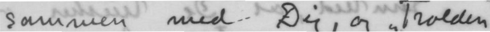sammen med Dig, og Trolden
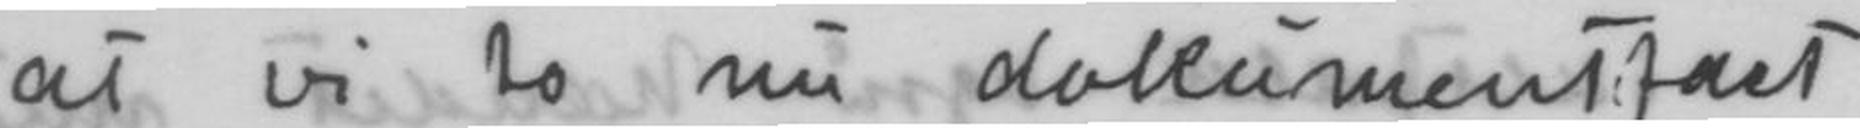at vi nu dokumentfast
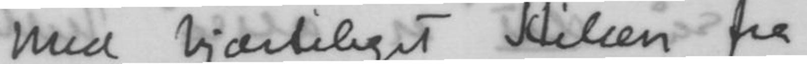Med hjærteliget Hilsen fra
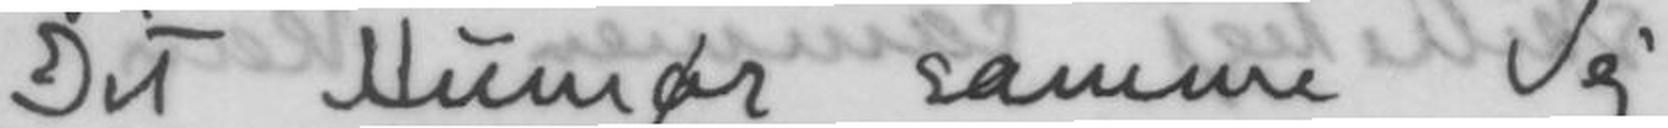Dit Humør samme Vej,
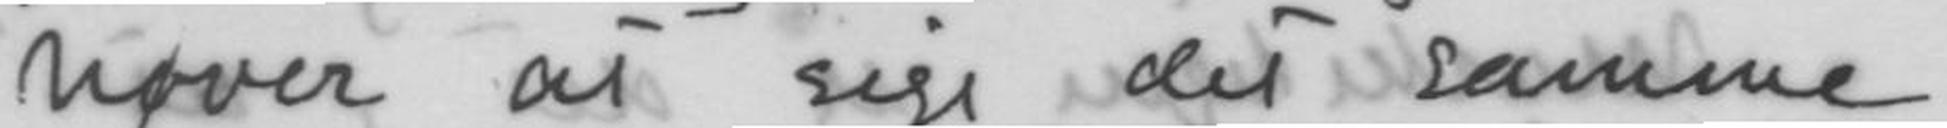høver at sige det samme
